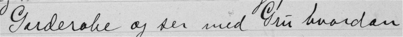Garderobe og ser med Gru hvordan
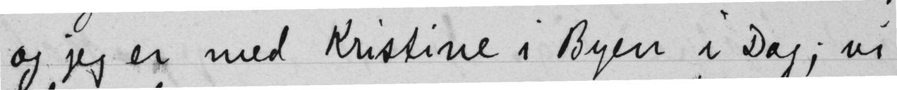og jeg er med Kristine i Byen i Dag; vi
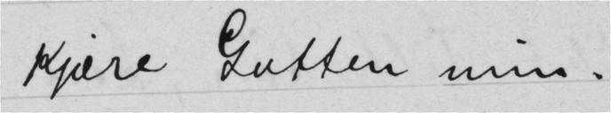Kjære gutten min.
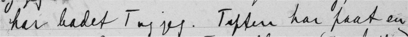har badet T og jeg. Tuften har faat en
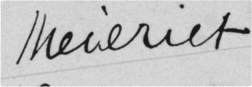Meieriet
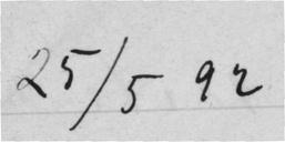25/5 92
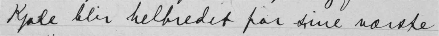Kjole blir helbredet for sine værste
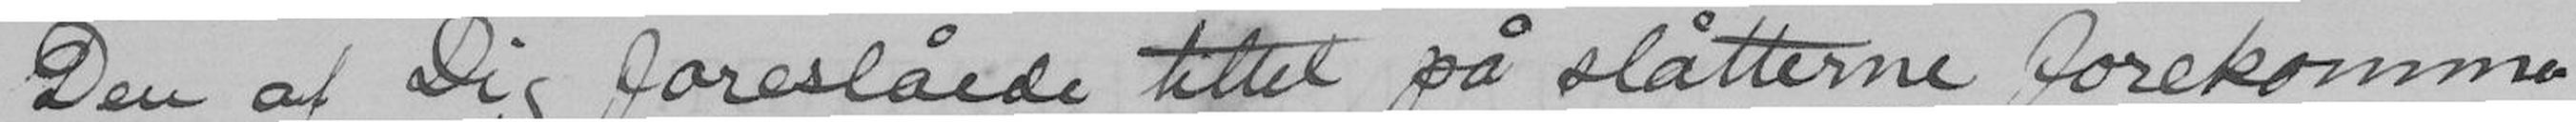Den af Dig foreslåede tittel på slåtterne forekommer
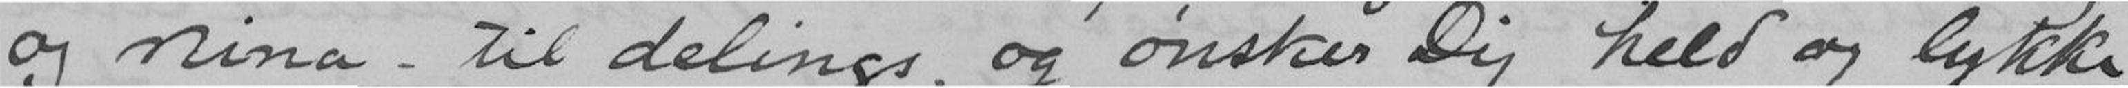og Nina - til delings, og ønsker Dig held og lykke
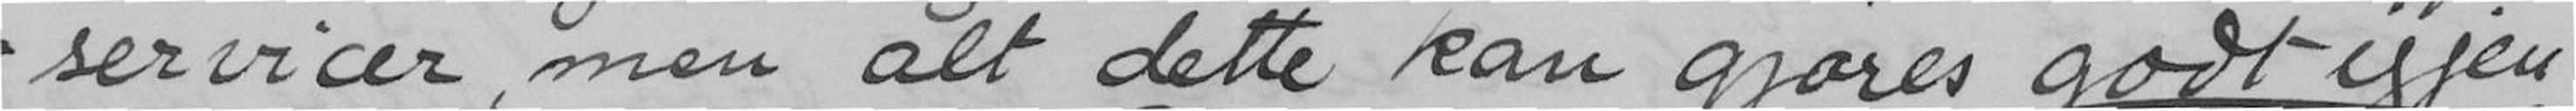servicer, men alt dette kan gjøres godt igjen
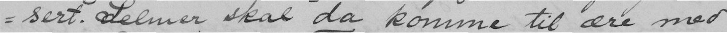sert. Selmer skal da komme til ære med
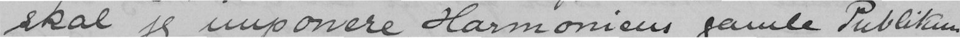skal jeg imponere Harmoniens gamle Publikum
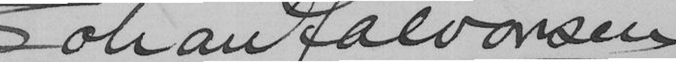Johan Halvorsen
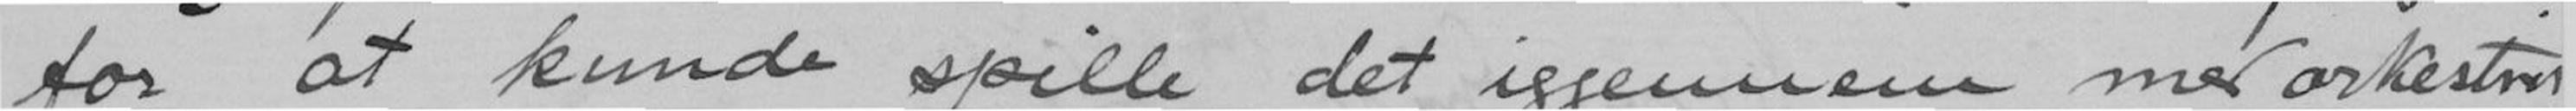for at spille det igjennem med orkesteret.
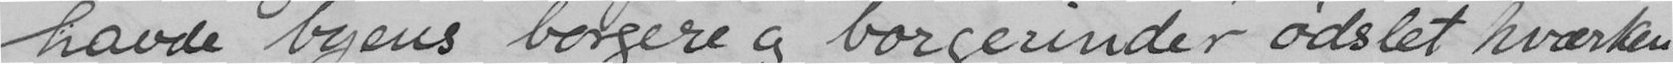havde byens borgere og borgerinder ødslet hværken
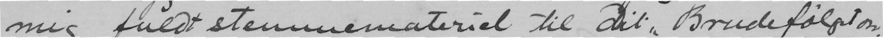mig fuldt stemmemateriel til Dit "Brudefølget osv.
